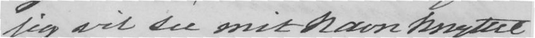jeg vil see mit Navn knyttet
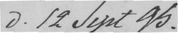12 Sept 95.
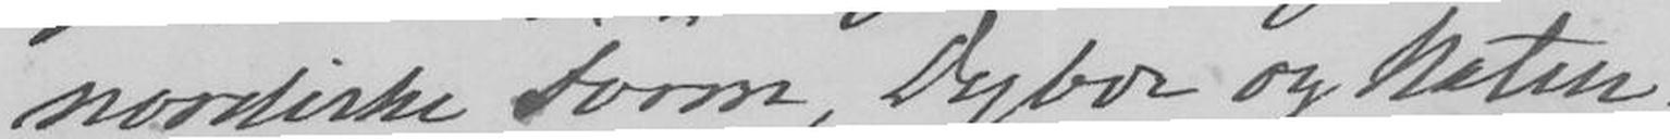nordiske Form, Dybde og Natur,
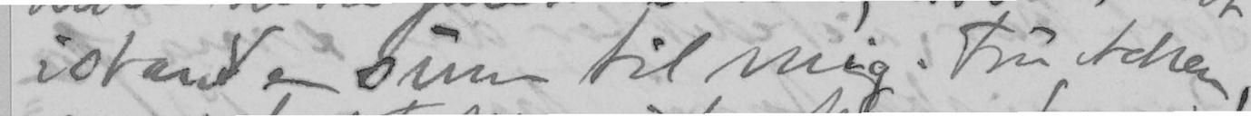istand en sum til mig. Fru Achen,
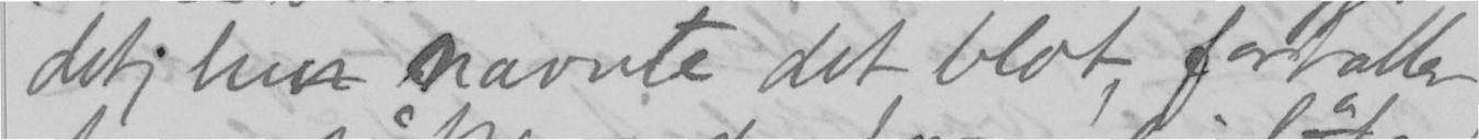det; hun nævnte det blot, fortæller
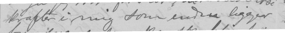kræfter i mig som endnu ligger
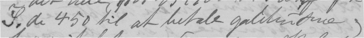S. de 450 til at betale galehusene,
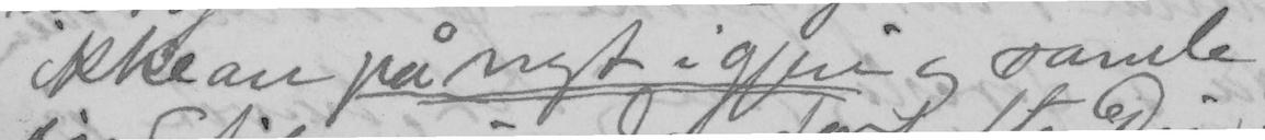ikke an på nyt igjen og samle
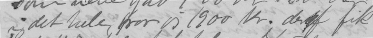i det hele,tro jeg 1900 kr. deraf fik
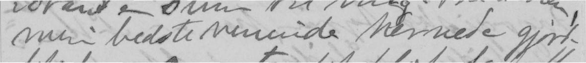min bedste veninde hernede gjorde
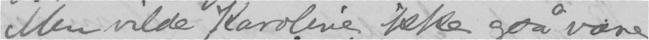Men vilde Karoline ikke også være
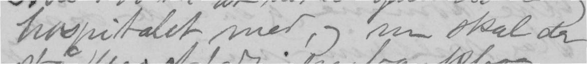hospitalet med, og nu skal der
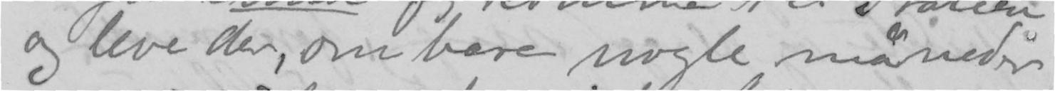og leve der, om bare nogle måneder
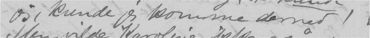jeg, kunde jeg komme derned!
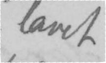lavet
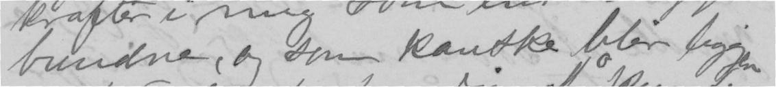bundne, og som kanske blir liggen
